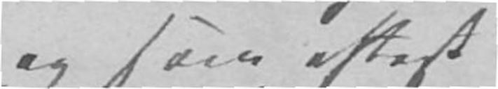og som oftest
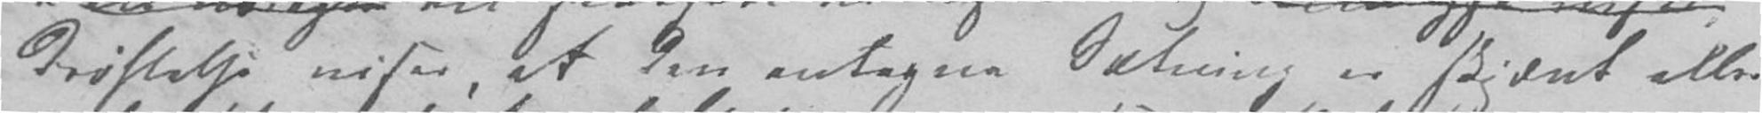Drøftelse viser, at den antagne Sætning er skjævt eller
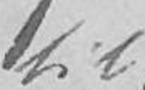til
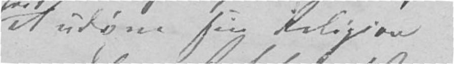at udøve sin Religion
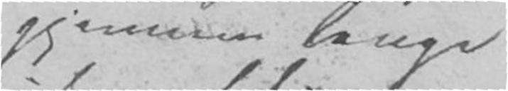gjennem lange
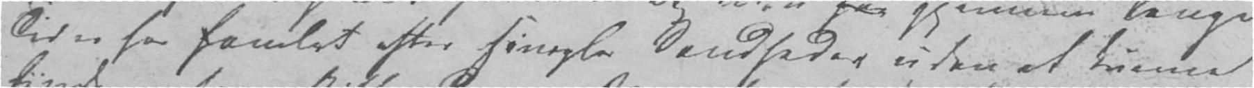Tider har famlet efter simple Sandheder uden at kunne
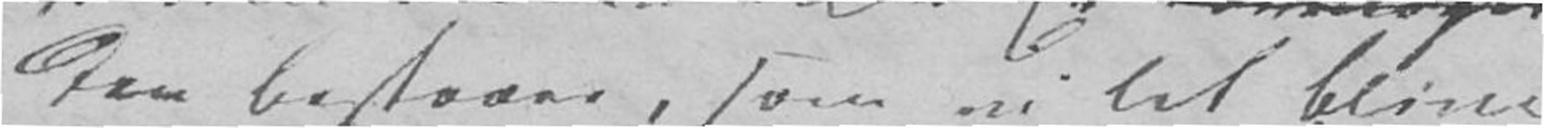Den bestaaer, som vi let bliver
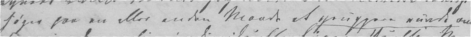søger paa en eller anden Maade at gruppere rundt om
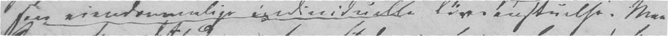sin eiendommelige individuelle Livsanskuelse. Men
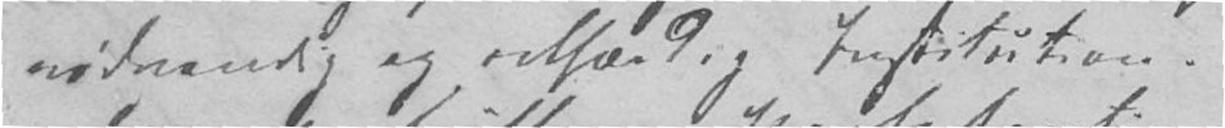nødvendig og retfærdig Institution.
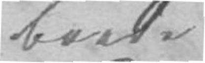baade
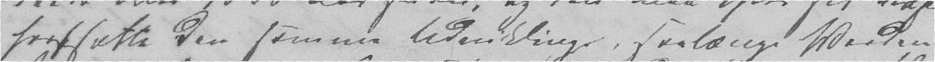fortsætte den samme Udvikling, saalænge Verden
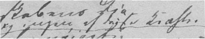og ingen af disse Kræfter
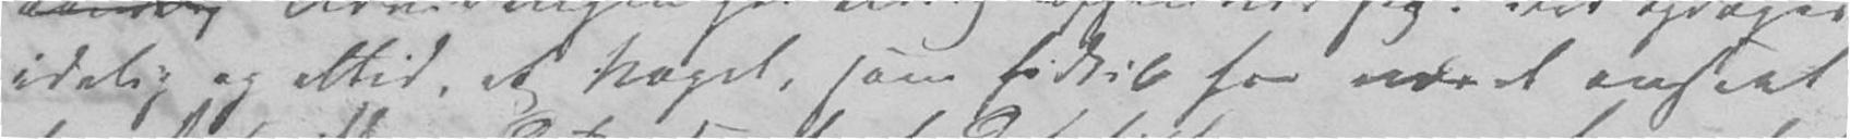idelig og altid, at Noget, som hidtil har været anseet
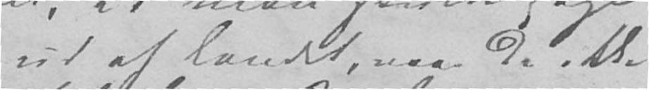ud af Landet, naar de ikke
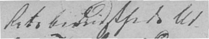Retsbevidstheds Ud-
